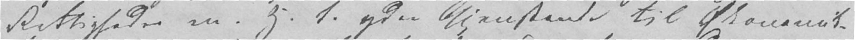Rettigheder m. H. t. ydre Gjenstande til Økonomik-
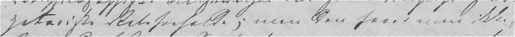gatoriske Retsforholde; men den faar man ikke.
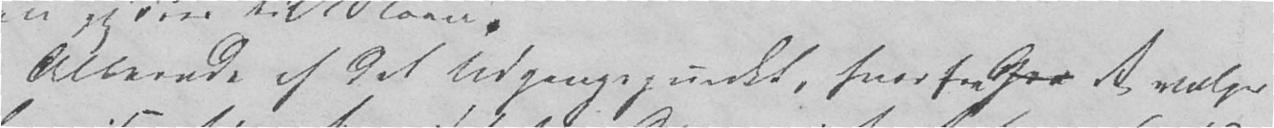Allerede af det Udgangspunkt, hvorfor de vælger
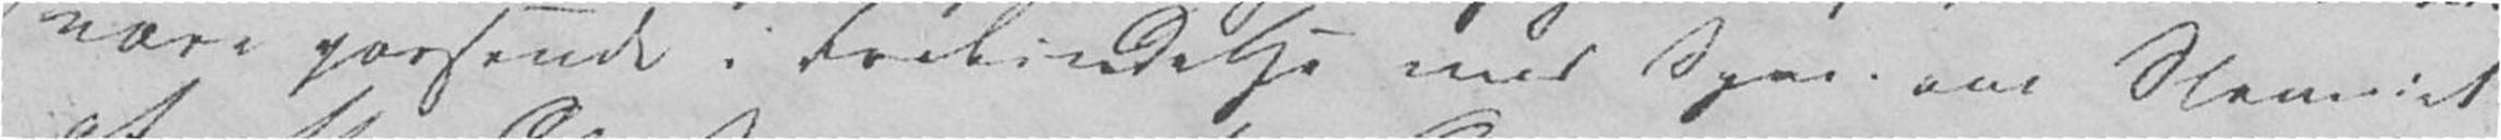være passende i Forbindelse med Spm. om Slaveriet
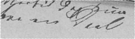for en Deel
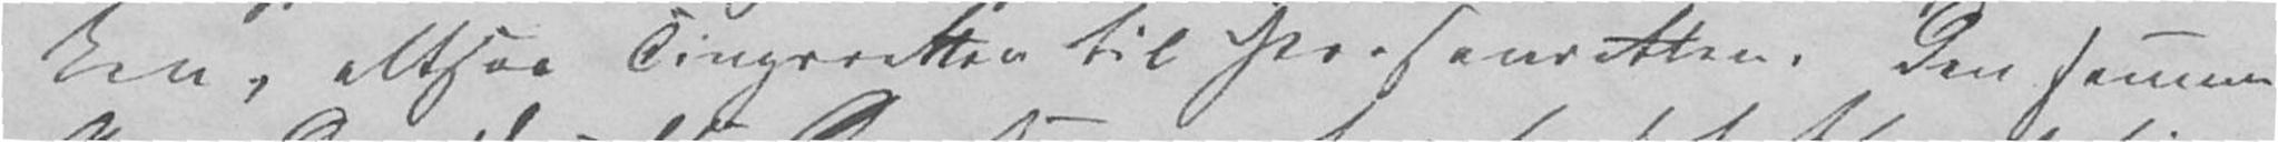ken, altsaa Tingsretten til Personretten. Den samme
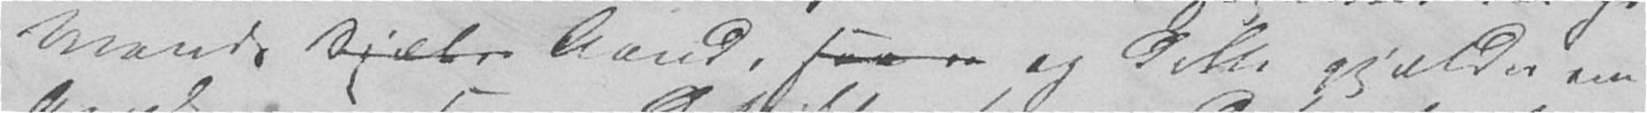Mands  Aand, og dette gjælder om
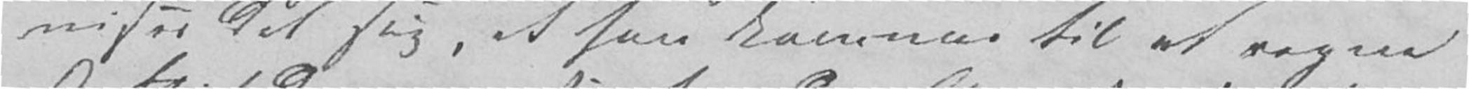viser det sig, at han kommer til at regne
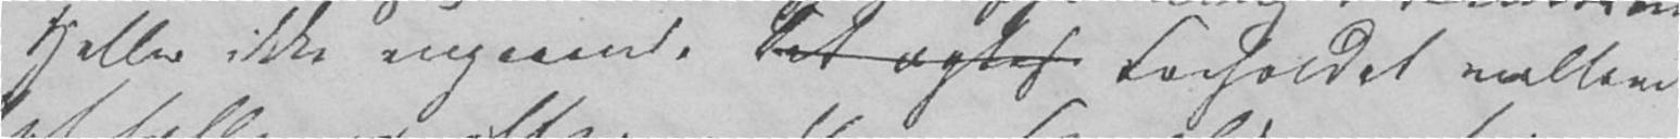Heller ikke angaaende  Forholdet mellem
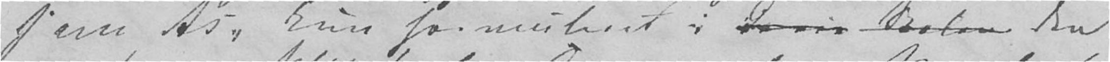som As; kun formuleret i den
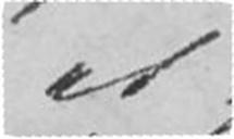at
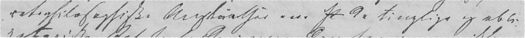retsphilosophiske Anskuelser om R de tinglige og obli-
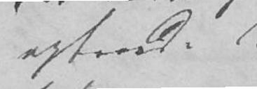opterede
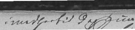imidlertid da kun
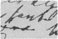hævde
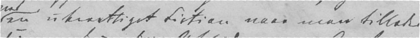en uberettiget Fiction naar man tillade
